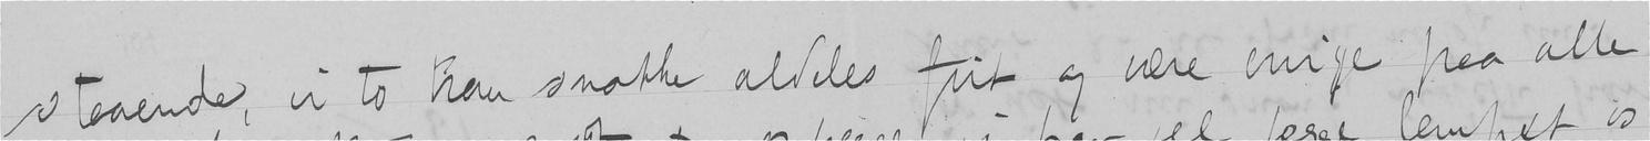staaende, vi to kan snakke aldeles frit og være enige paa alle
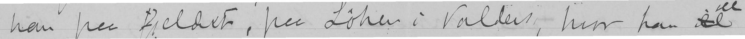han paa fjeldet, paa Løken i Valders, hvor han vil
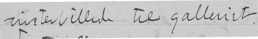vinterbillede til galleriet.
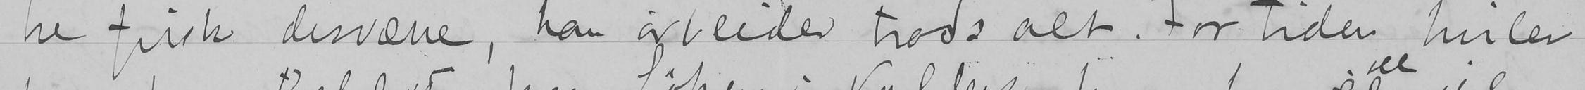ke frisk desværre, han arbeider trods alt. For tiden hviler
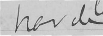havde
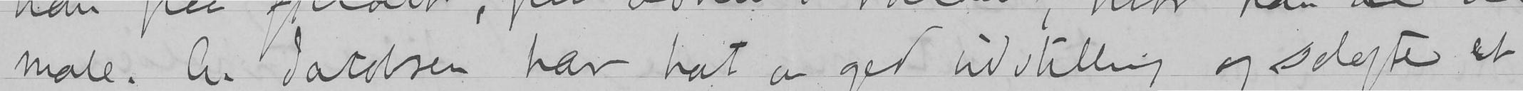male. A. Jacobsen har hat en god udstilling og solgte et
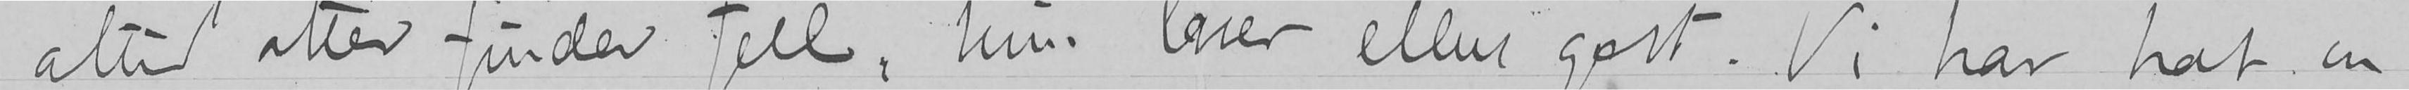altid atter finder feil, hun lever ellers godt. Vi har hat en
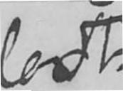bedt
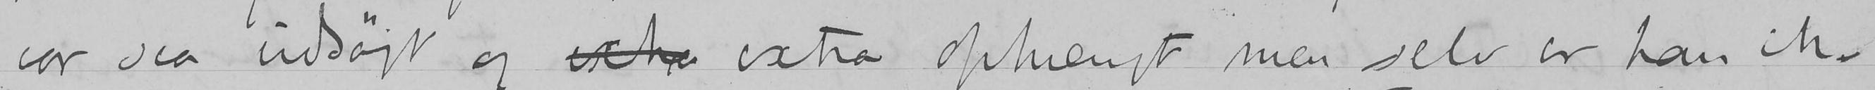var saa udsøgt og extra extra ophængt men selv er han ik-
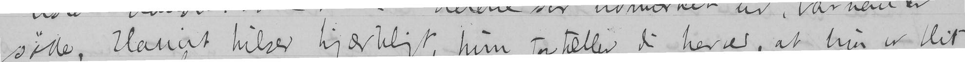søde, Harriet hilser hjærteligt, hun fortæller dig herved, at hun er blit
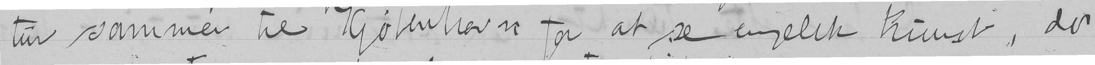tur sammen til Kjøbenhavn for at se engelsk kunst, det
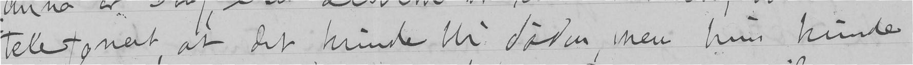telefonert, at det kunde bli døden, men hun kunde
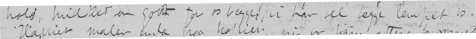hold, hvilket er godt for os begge, vi har vel begge lempet os.
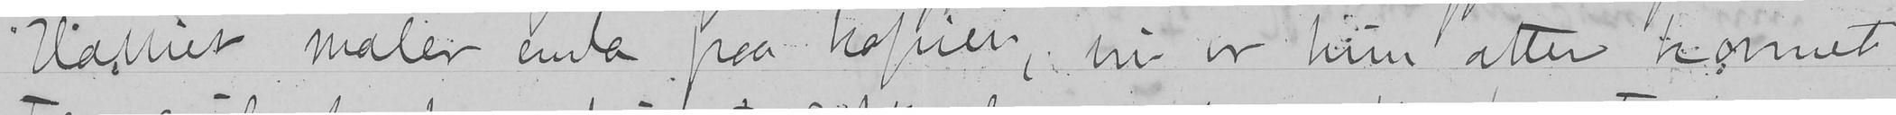Harriet maler enda paa kopien, nu er hun atter kommet
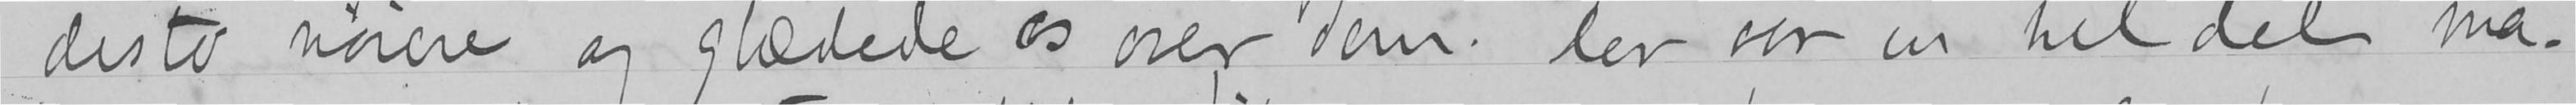desto nøiere og glædede os over dem. Der var en hel del ma-
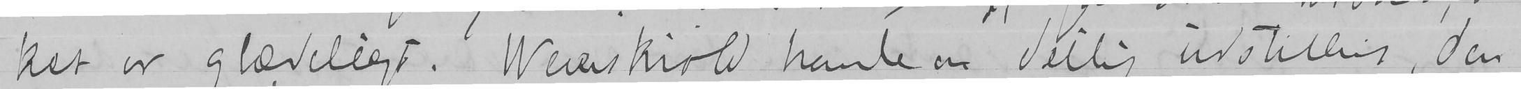ket er glædeligt. Werenskiold havde en deilig udstilling, den
